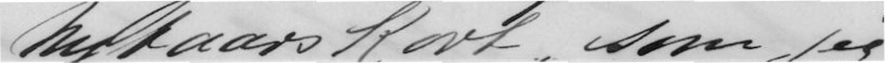Nytaarskort, som jeg
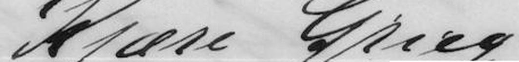Kjære Grieg!
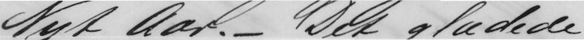Nyt Aar. - Det glædede
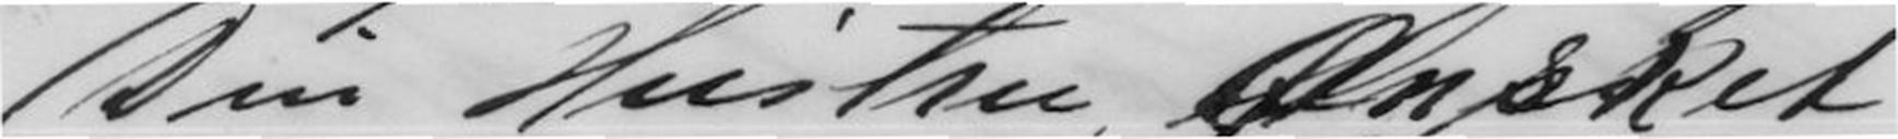Din Hustru Ønsket
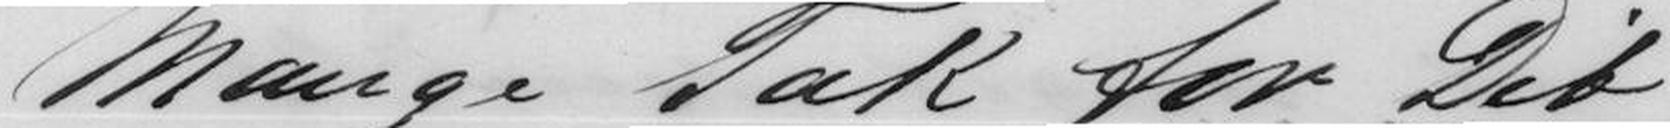Mange Tak for Dit
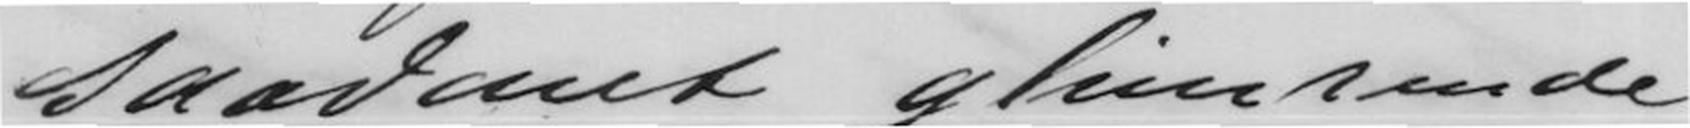saadant glimrende
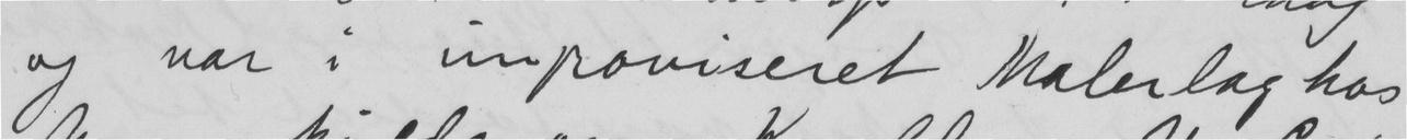og var i improviseret Malerlag hos
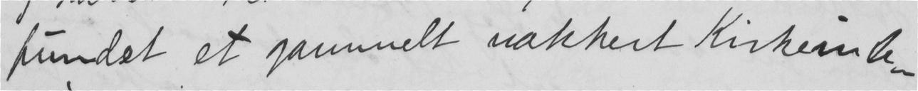fundet et gammelt vakkert Kirkeinte-
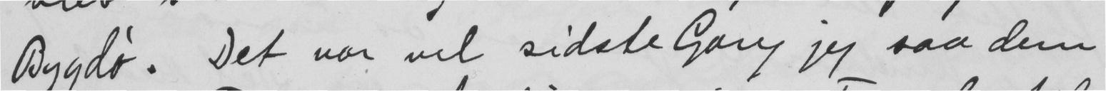Bygdø. Det var vel sidste Gang jeg saa dem
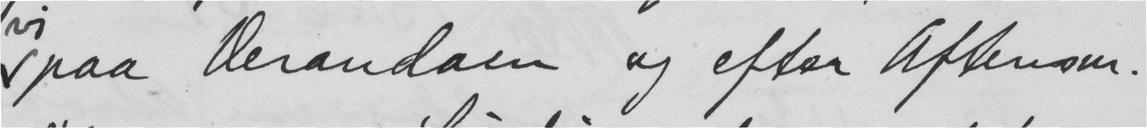paa Verandaen og efter Aftensen.
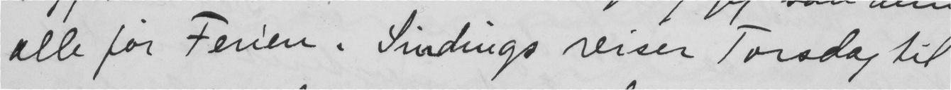alle før Ferien. Sindings reiser Torsdag til
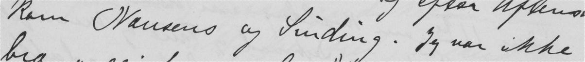kom Nansens og Sinding. Jeg var ikke
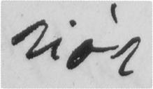riør
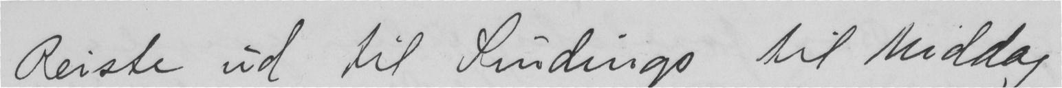Reiste ud til Sindings til Middag
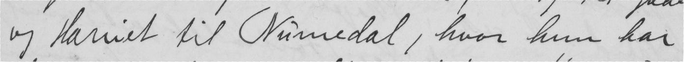og Harriet til Numedal, hvor hun har
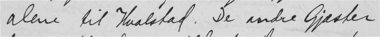alene til Hvalstad. De andre Gjæster
